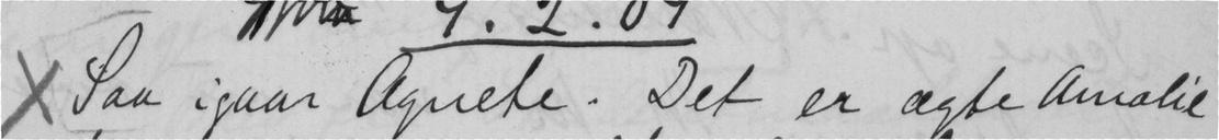x Saa igaar Agnete. Det er ægte Amalie
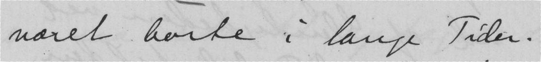været borte i lange Tider.
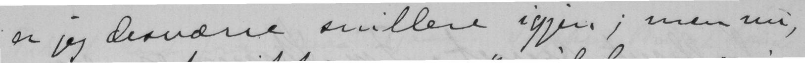er jeg desværre snillere igjen; men nu,
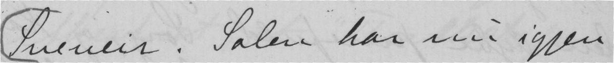Sneveir. Solen har nu igjen
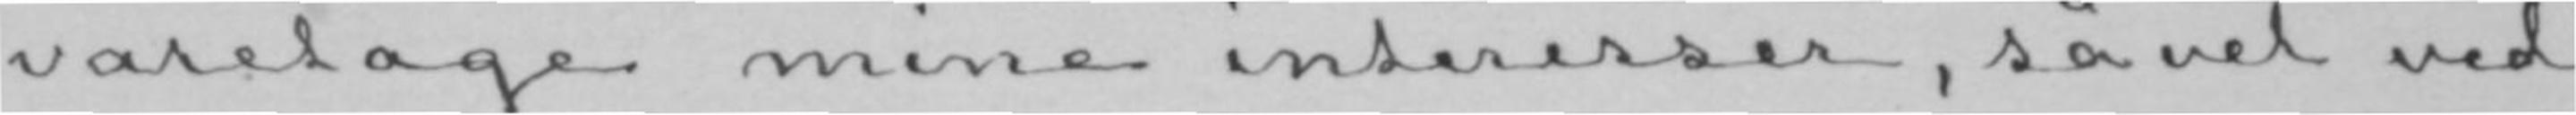varetage mine interesser, såvel ved
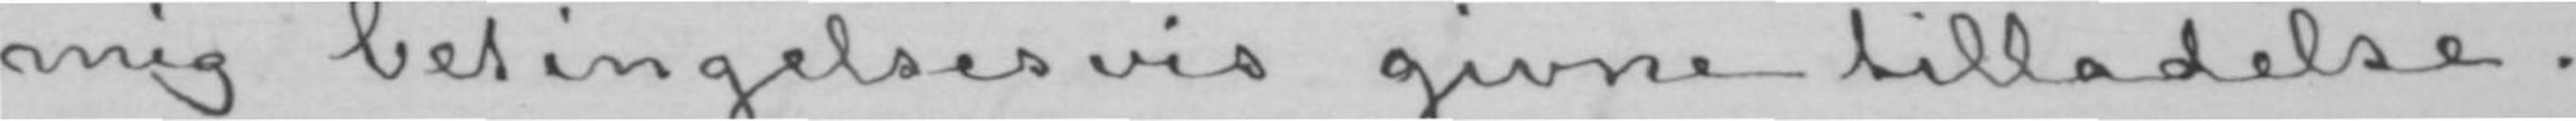mig betingelsesvis givne tilladelse.
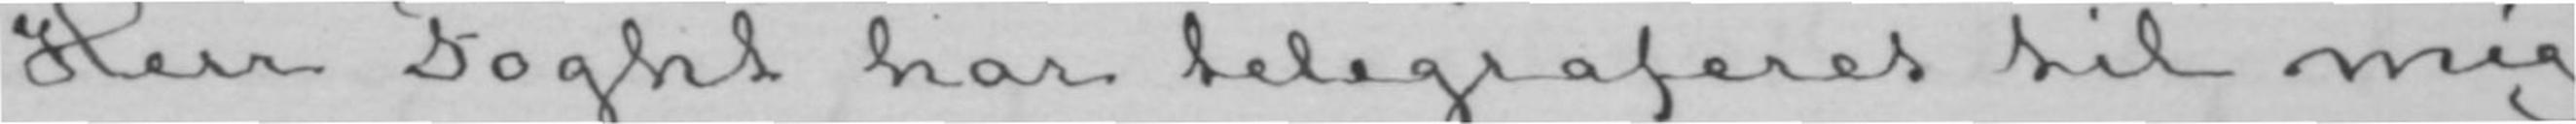Herr Foght har telegraferet til mig
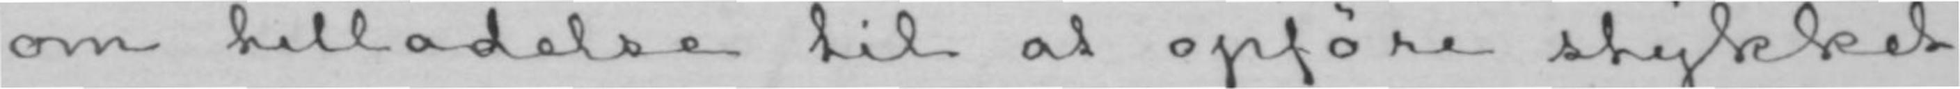om tilladelse til at opføre stykket
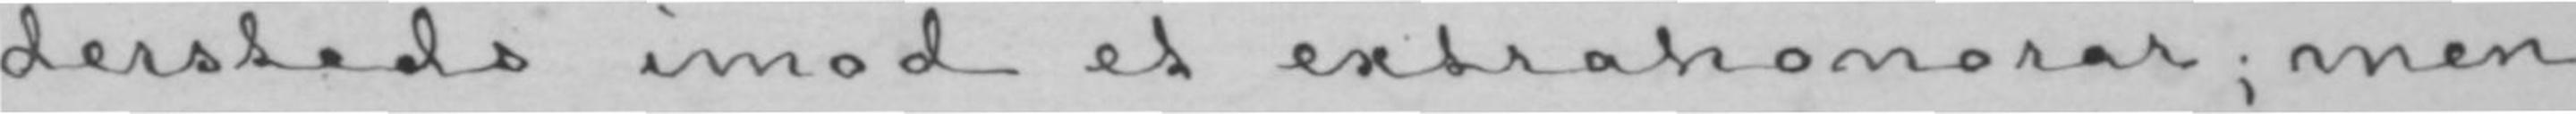dersteds imod et extrahonorar; men
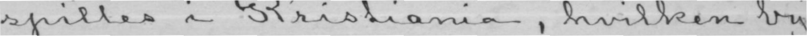spilles i Kristiania, hvilken by
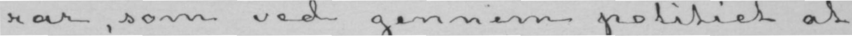rar, som ved gennem politiet at
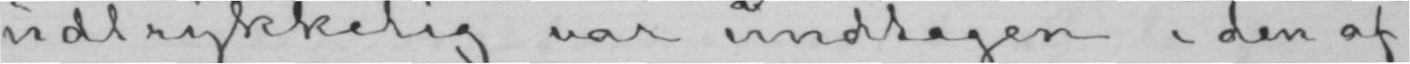udtrykkelig var undtagen i den af
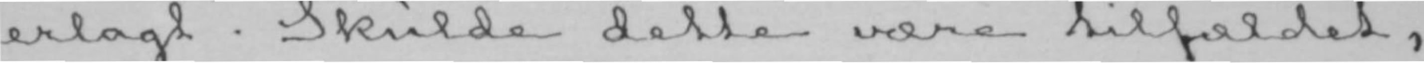erlagt. Skulde dette være tilfældet,
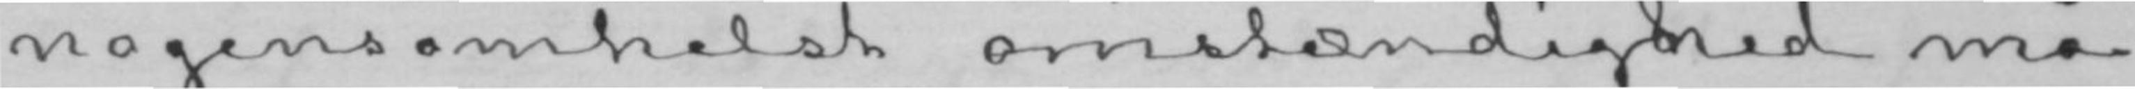nogensomhelst omstændighed må
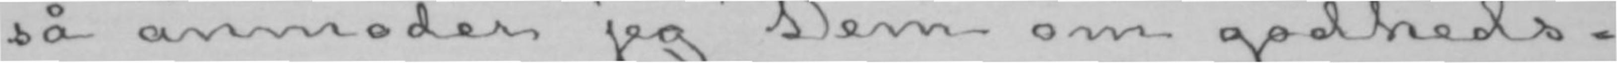så anmoder jeg Dem om godheds-
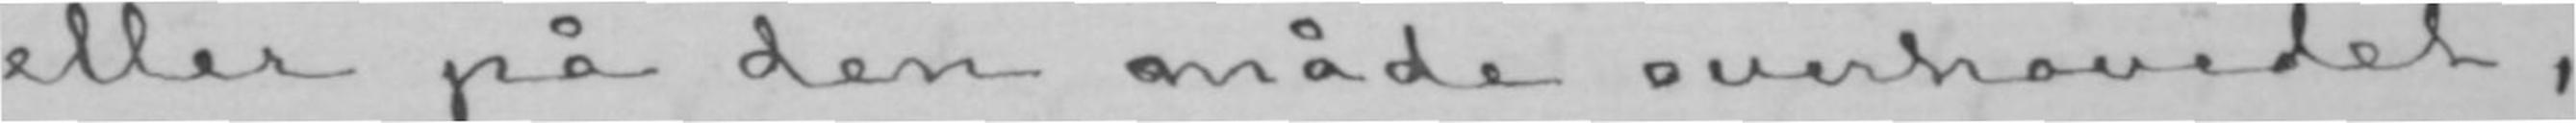eller på den måde overhovedet,
